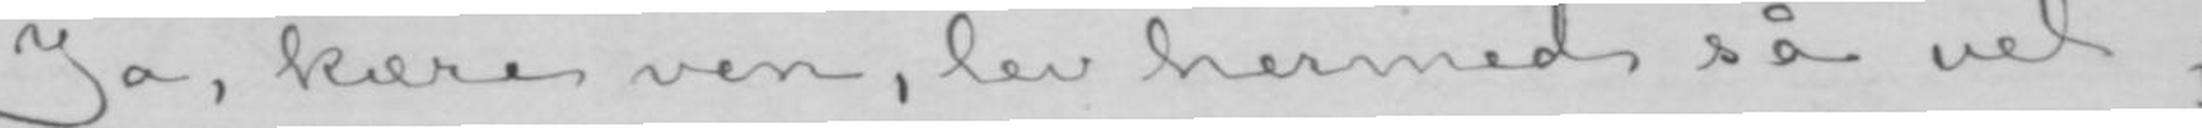Ja, kære ven, lev hermed så vel;
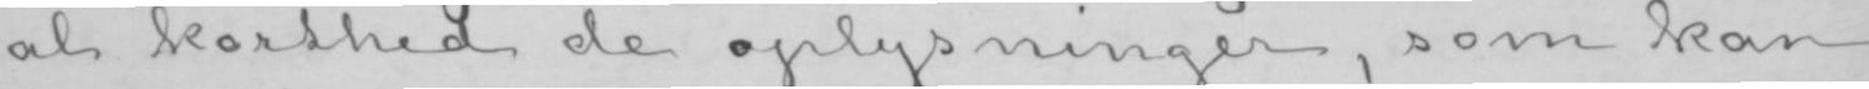al korthed de oplysninger, som kan
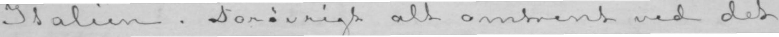Italien. Forøvrigt alt omtrent ved det
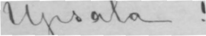Upsala!
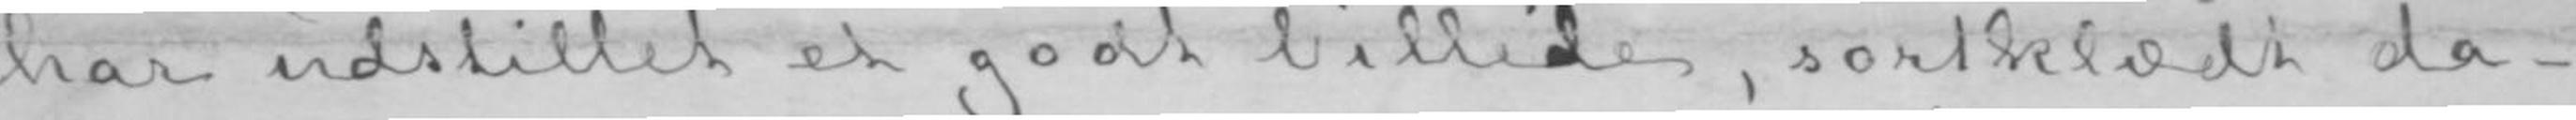har udstillet et godt billede, sortklædt da-
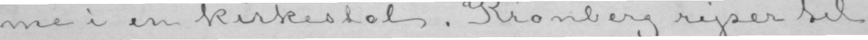me i en kirkestol. Kronberg rejser til
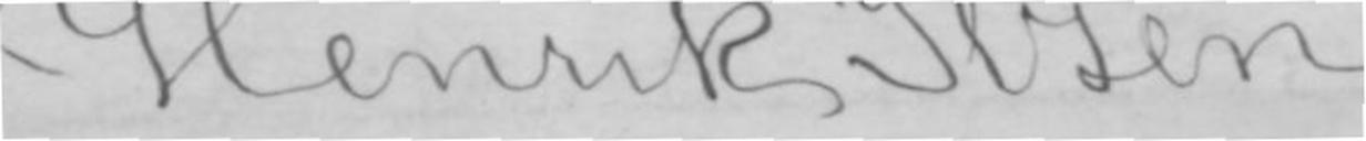Henrik Ibsen.
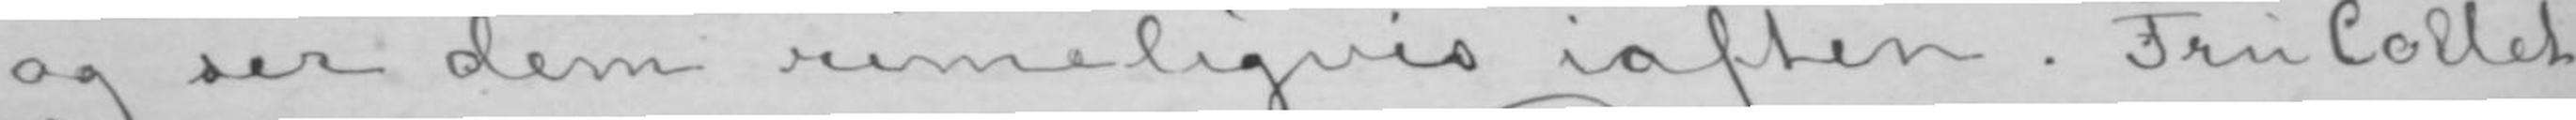og ser dem rimeligvis iaften. Fru Collet
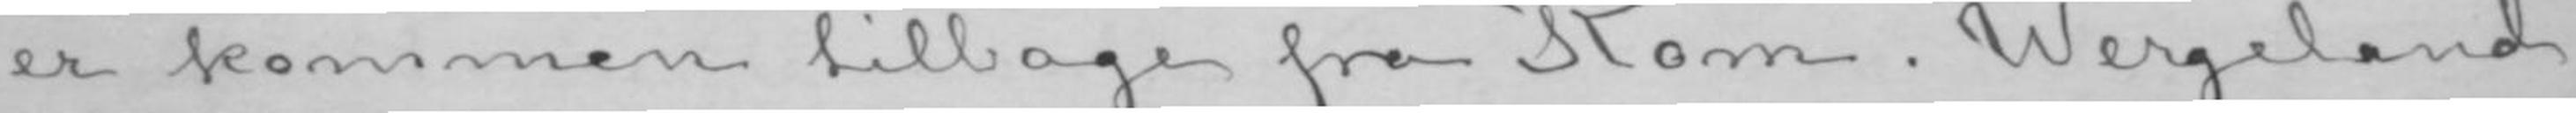er kommen tilbage fra Rom. Wergeland
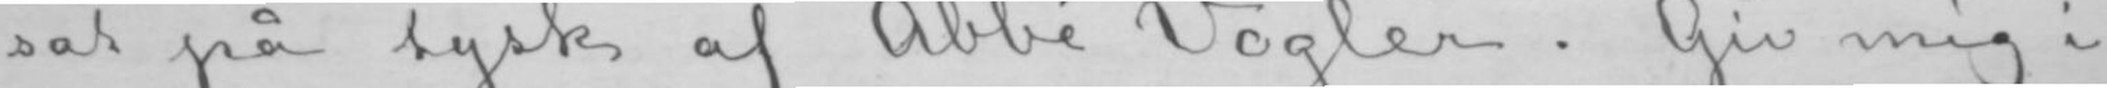sat på tysk af Abbè Vogler. Giv mig i
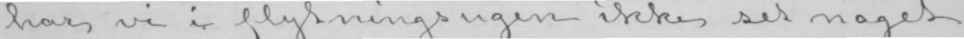har vi i flytningsugen ikke set noget
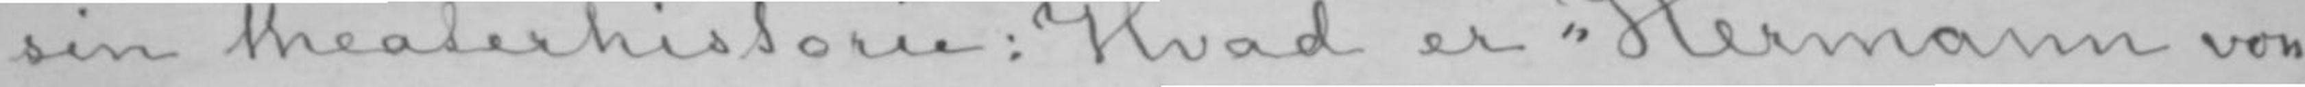sin theaterhistorie: Hvad er «Hermann von
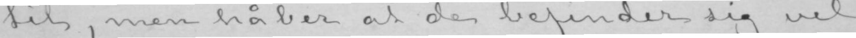til, men håber at de befinder sig vel,
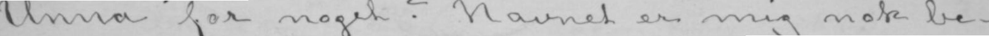Unna» for noget? Navnet er mig nok be-
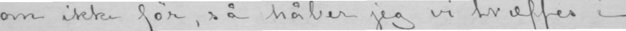om ikke før, så håber jeg vi træffes i
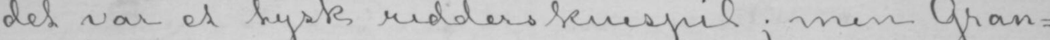det var et tysk ridderskuespil; men Gran-
